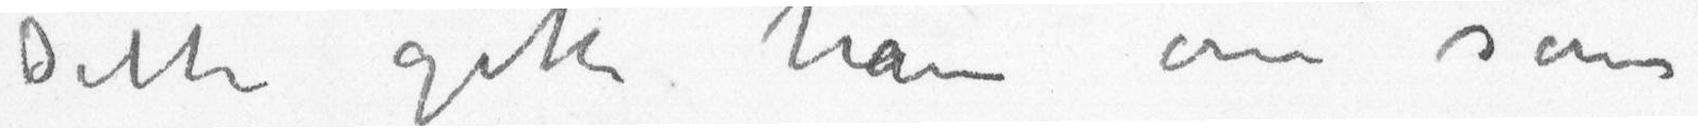Dette gik han om som
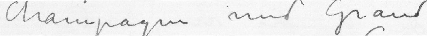Champagne med Grand
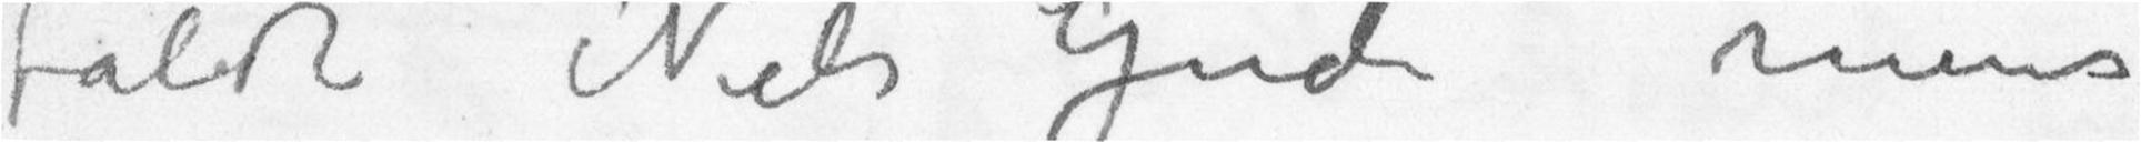faldt Nils Gude mens
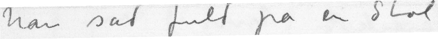han sad fuld på en Stol
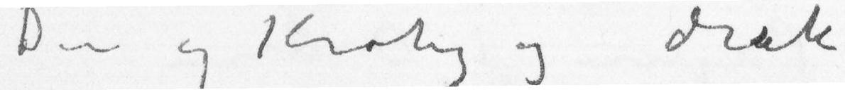Du og Krohg og drak
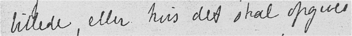billede, eller hvis det skal opgives
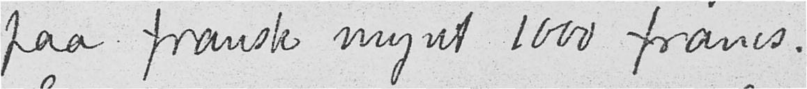paa fransk mynt 1000 francs.
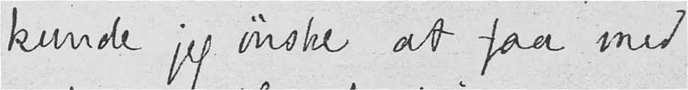kunde jeg ønske at faa med
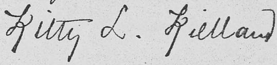Kitty L. Kielland
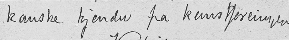kanske kjender fra kunstforeningen.
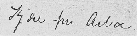Kjære fru Arbo!
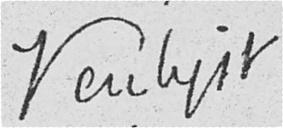Venligst
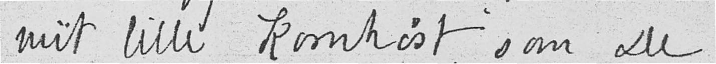mit lille "Kornhøst", som De
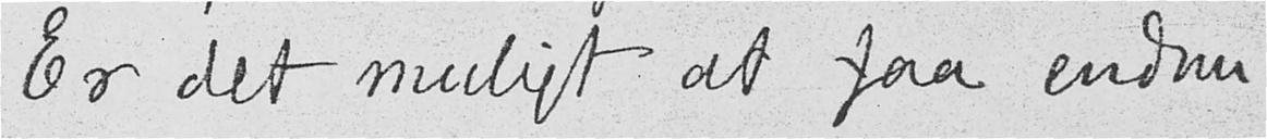Er det muligt at faa endnu
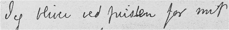Jeg bliver ved prisen for mit
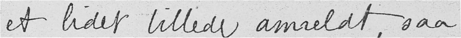et lidet billede anmeldt, saa
